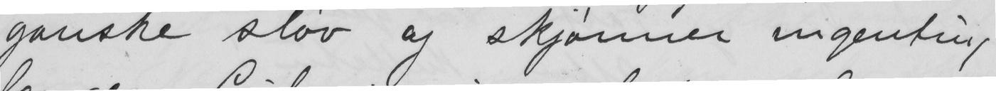ganske sløv og skjønner ingenting
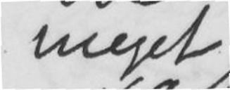meget
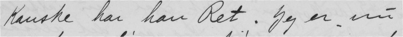Kanske har han Ret. Jeg er nu
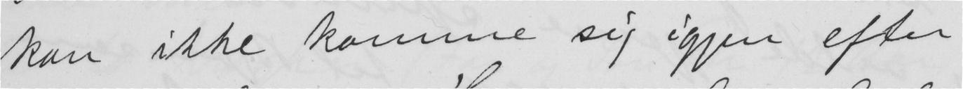kan ikke komme sig igjen efter
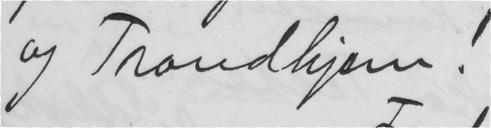og Trondhjem!
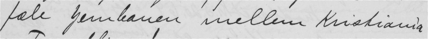fæle Jernbanen mellem Kristiania
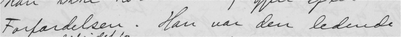Forfærdelsen. Han var den ledende
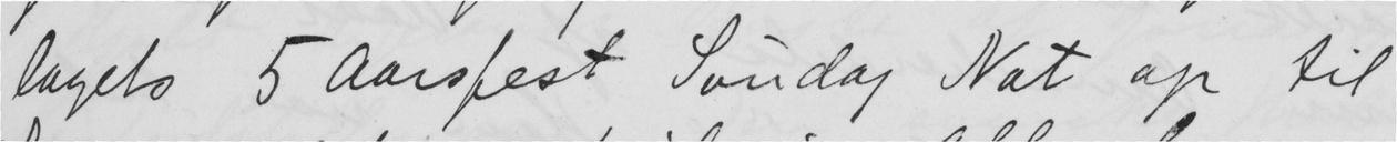lagets 5 Aarsfest Søndag Nat op til
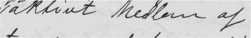aktivt Medlem af
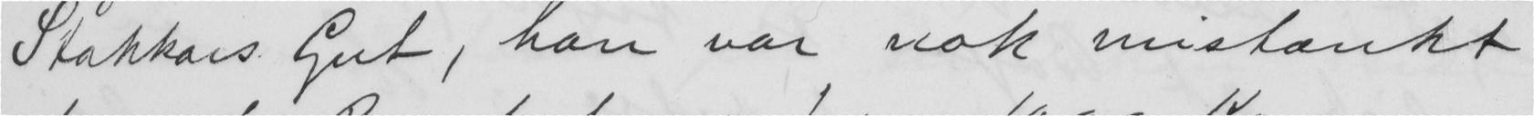Stakkars Gut, han var nok mistænkt
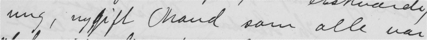ung, nygift Mand som alle var
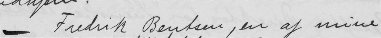- Fredrik Bentsen, en af mine
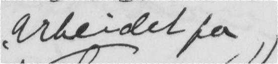arbeidet for
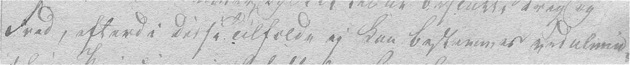Fred, efterdi disse Tilfælde ey kan bestemmes ved almin-
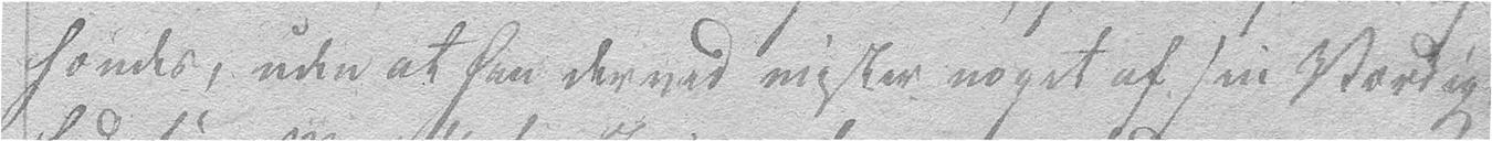hændes, uden at han derved mister noget af sin Værdig-
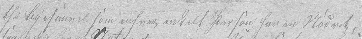thi ligesaavel som enhver enkelt Person har en Nødret,
